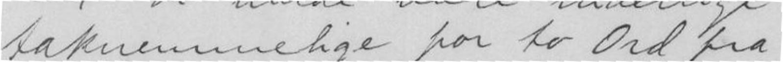taknemmelige for to Ord fra
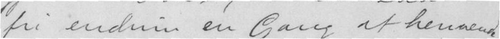fri endnu en Gang at henvende
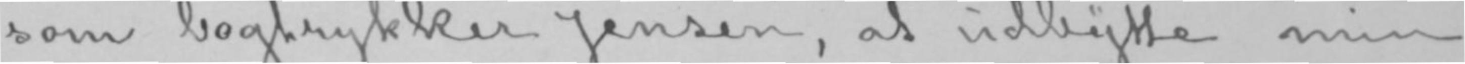som bogtrykker Jensen, at udbytte min
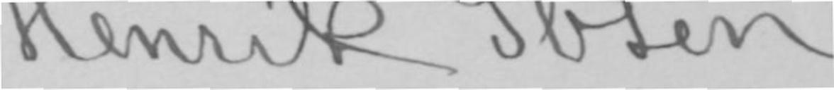Henrik Ibsen.
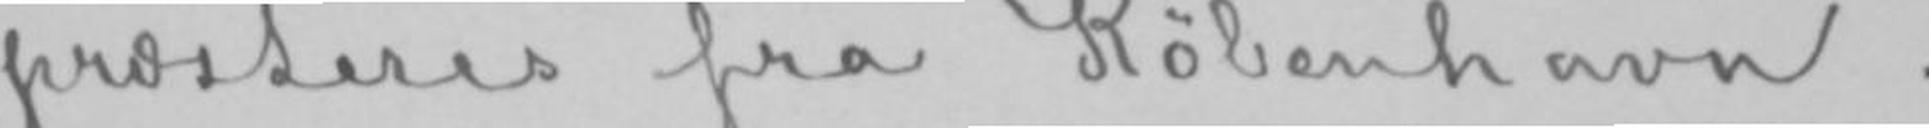præsteres fra København.
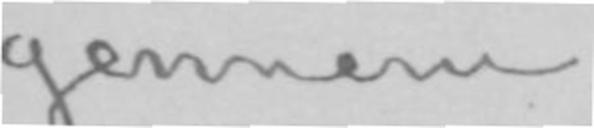gennem
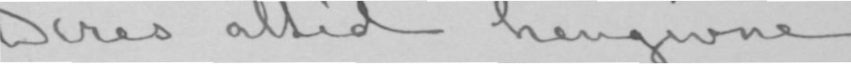Deres altid hengivne
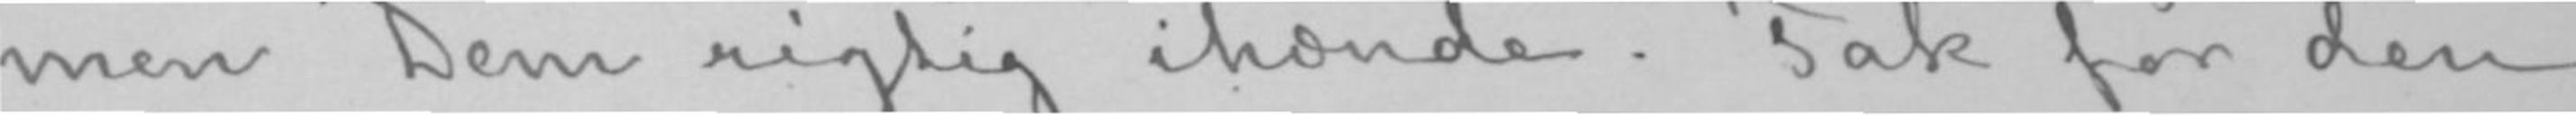men Dem rigtig ihænde. Tak for den
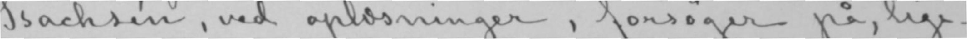Isachsen, ved oplæsninger, forsøger på, lige-
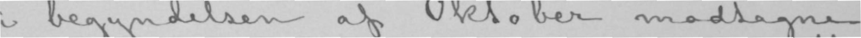i begyndelsen af Oktober modtagne
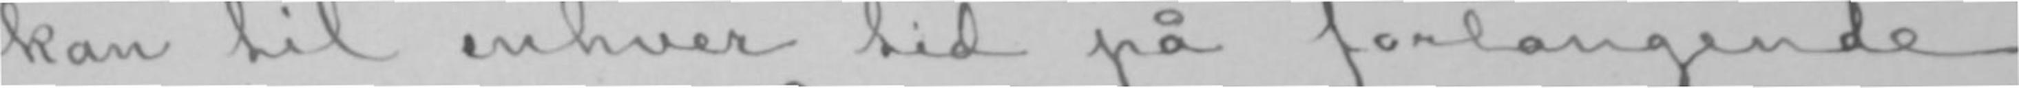kan til enhver tid på forlangende
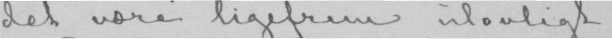det være ligefrem ulovligt.
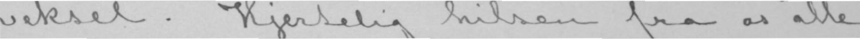veksel. Hjertelig hilsen fra os alle
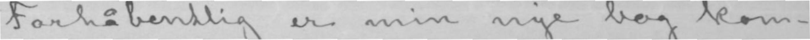Forhåbentlig er min nye bog kom-
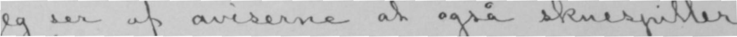Jeg ser af aviserne at også skuespiller
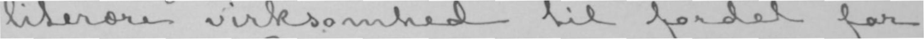literære virksomhed til fordel for
